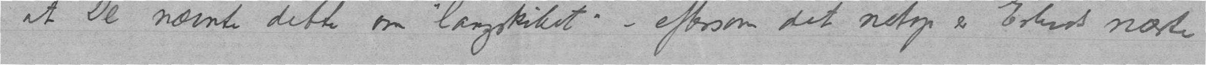at De nævnte dette om «langskibet» – eftersom det netop er Erlends næste
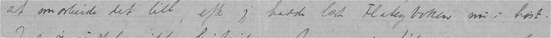at omarbeide det litt, efter jeg hadde læst Flateyboken nu i høst.
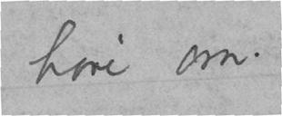høre om.
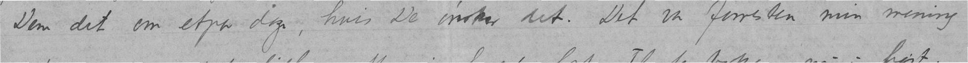Dem det om etpar dager, hvis De ønsker det. Det var forresten min mening
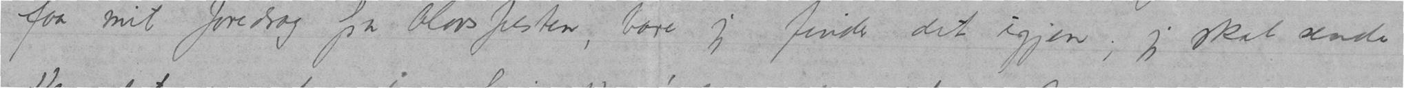faa mit foredrag fra Olavsfesten, bare jeg finder det igjen; jeg skal sende
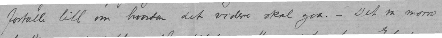fortælle litt om hvordan det videre skal gaa. – Det var morro
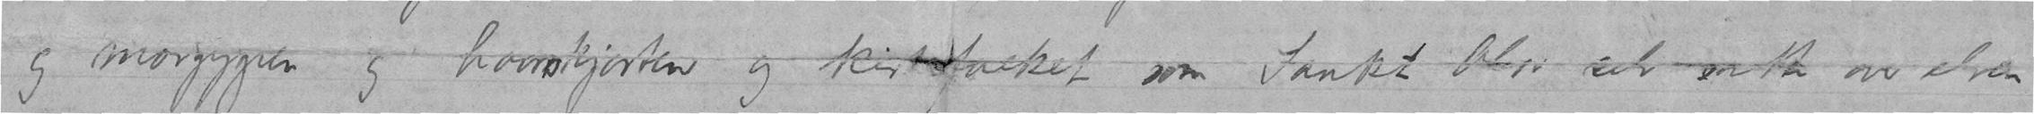og margygren og haarskjorten og kistefolket som Sankt Olav selv satte over elven
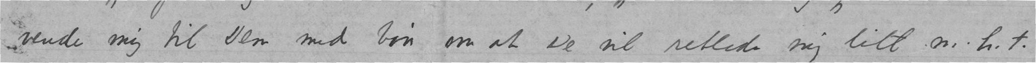vende mig til Dem med bøn om at De vil retlede mig litt m.h.t.
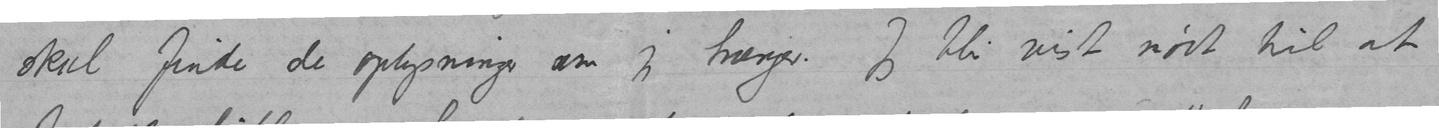skal finde de oplysninger som jeg trænger. Jeg blir vist nødt til at
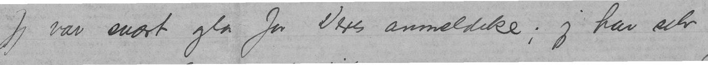Jeg var svært gla for Deres anmeldelse; jeg har selv
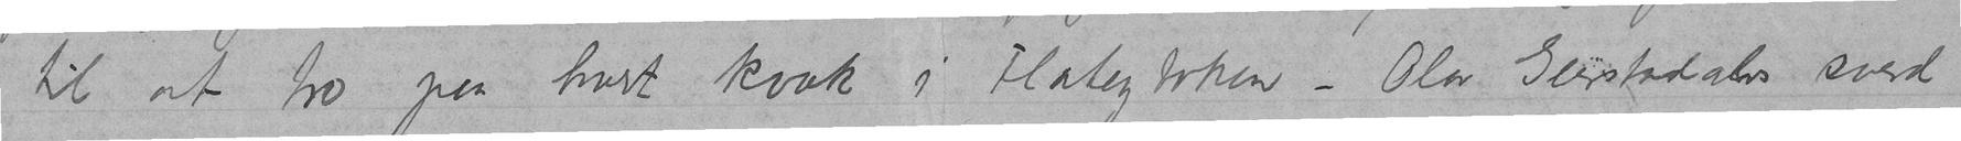til at tro paa hvert kvæk i Flateyboken – Olav Geirstadalvs sverd
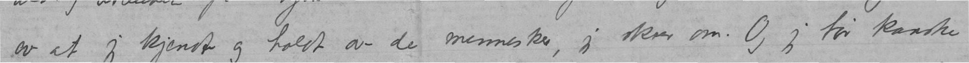av at jeg kjendte og holdt av de mennesker, jeg skrev om. Og jeg tør kanske
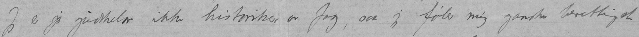Jeg er jo gudskelov ikke historiker av fag, saa jeg føler mig ganske berettiget
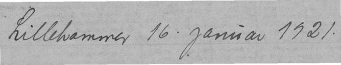Lillehammer 16. januar 1921.
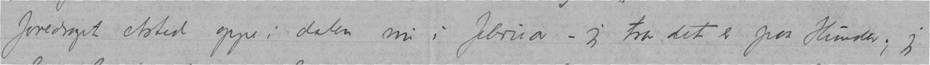foredraget etsted oppe i dalen nu i februar – jeg tror det er paa Hunder; jeg
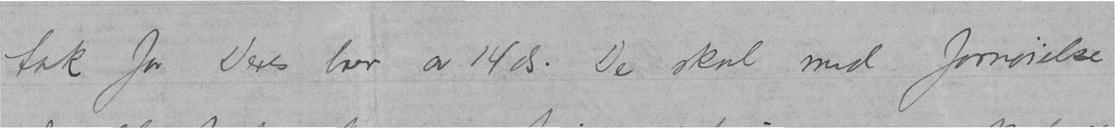tak for Deres brev av 14ds. De skal med fornøielse
